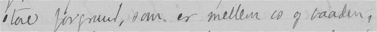store forgrund, som er mellem os og baaden,
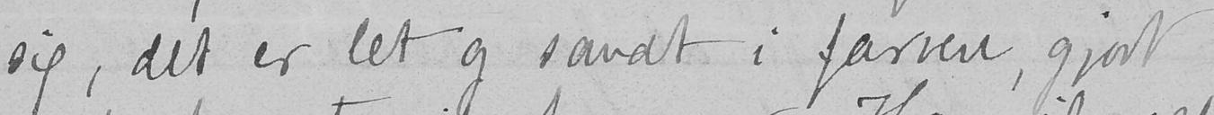sig, det er let og sandt i farven, gjort
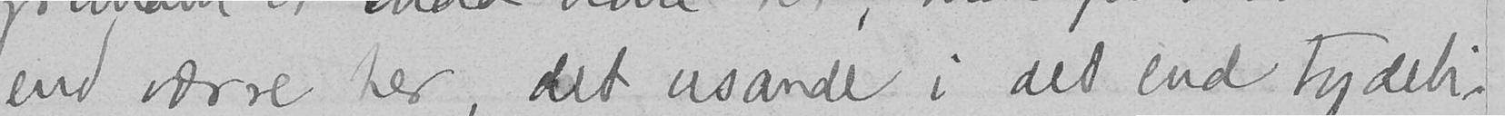end værre her, det usande i det end tydelig-
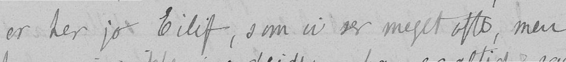er her jo Eilif, som vi ser meget ofte, men
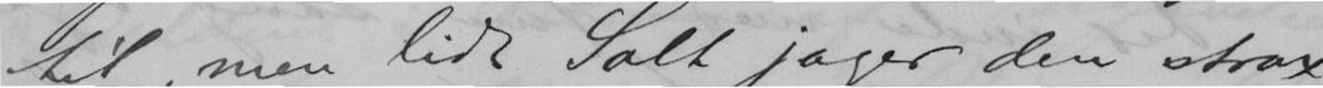til, men lidt Salt jager den strax
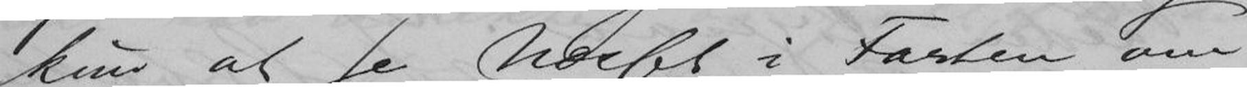kun at se Næsset i Farten om
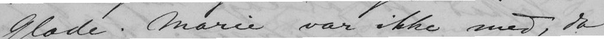Glæde. Marie var ikke med, da
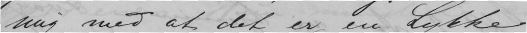mig med at det er en Lykke
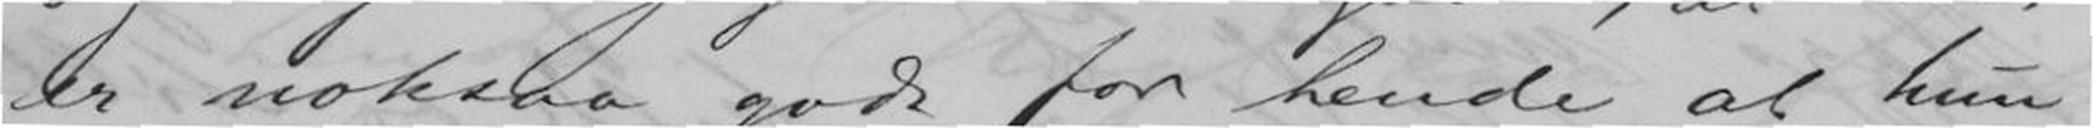er noksaa godt for hende, at hun
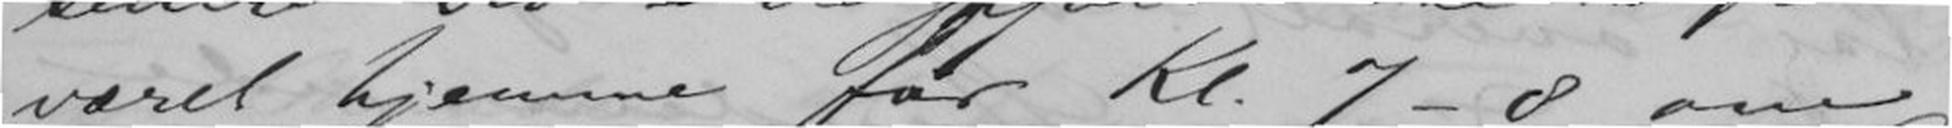været hjemme før Kl. 7 - 8 om
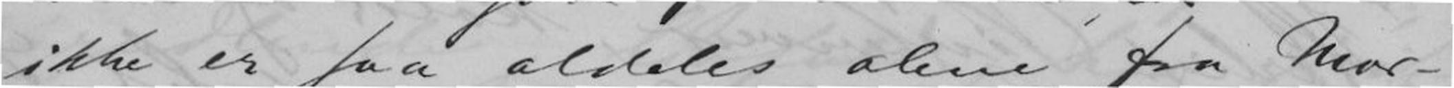ikke er saa aldeles alene fra Mor-
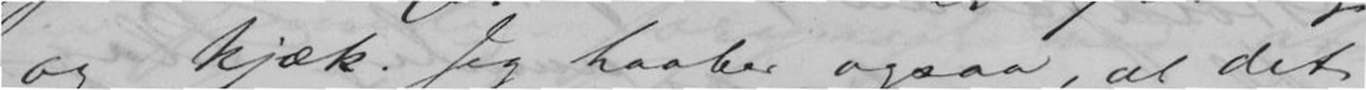og kjæk. Jeg haaber ogsaa, at det
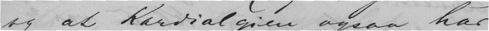og at Kardialgien ogsaa har
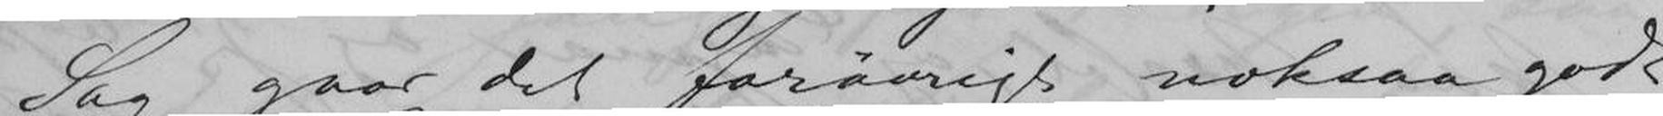Sag gaar det forøvrigt noksaa godt
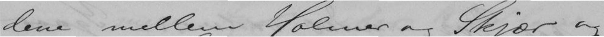dene mellem Holmer og Skjær og
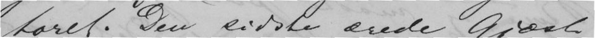toret. Den sidste ærede Gjæst
